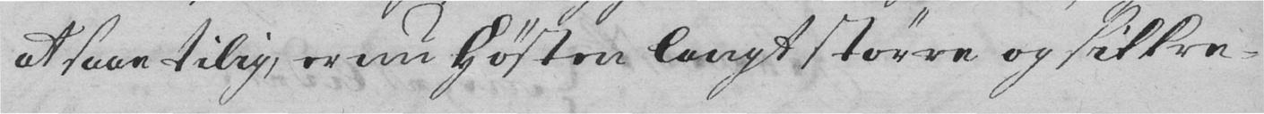at saae tilig, er nu Høsten langt større og sikkre-
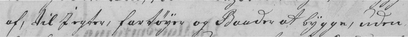af, til Iegter, fartøyer og Baader at bygge, uden
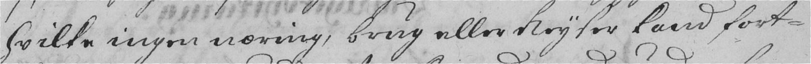hvilke ingen næring, brug eller Reyser kand fort-
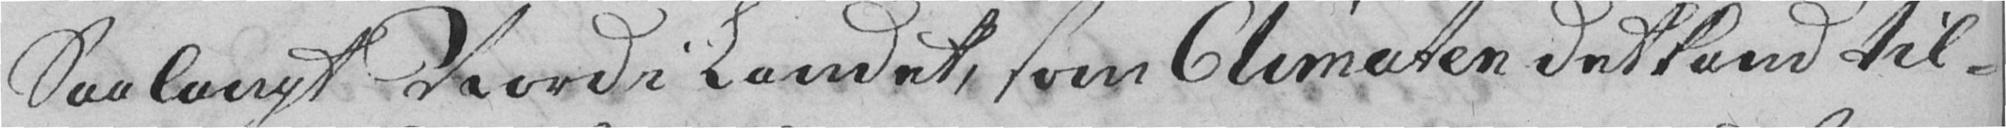Saalangt Nord i Landet, som Climaten det kand til-
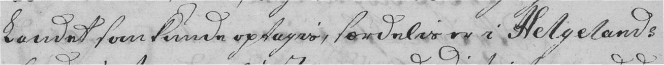Landet som kunde optagis, særdelis er i Helgelands
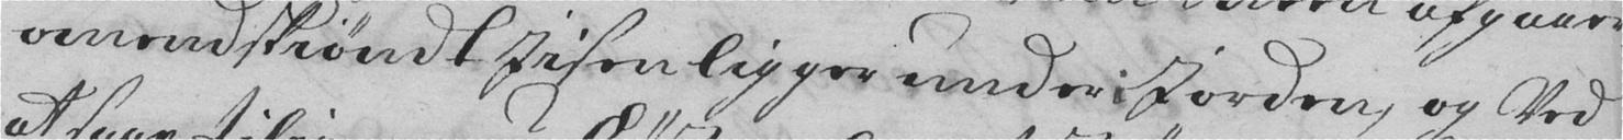omendskiøndt Iisen ligger under Iorden, og Ved
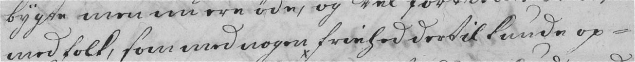med Folk, som med nogen friehed dertil kunde op-
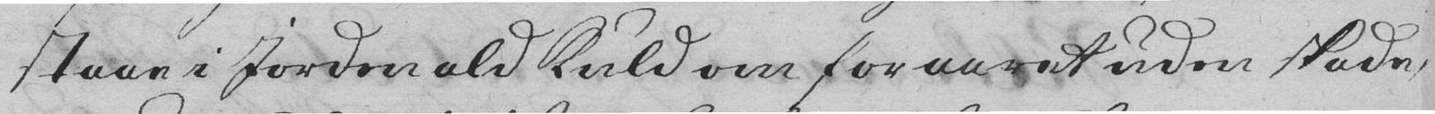staae i Iorden ald kuld om foraaret uden skade,
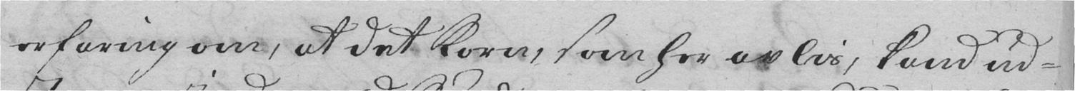erfaring om, at det Korn, som her avlis, kand ud-
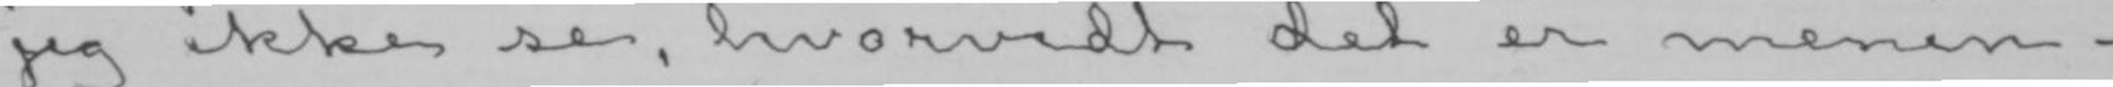jeg ikke se, hvorvidt det er menin-
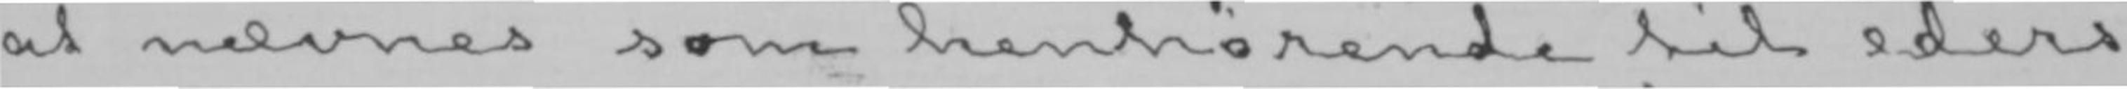at nævnes som henhørende til eders
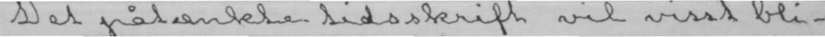Det påtænkte tidsskrift vil visst bli-
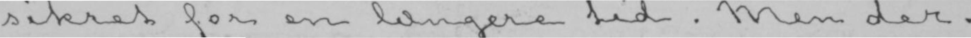sikret for en længere tid. Men der-
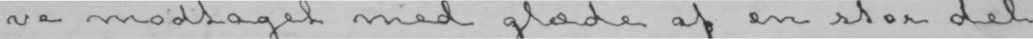ve modtaget med glæde af en stor del
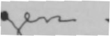gen.
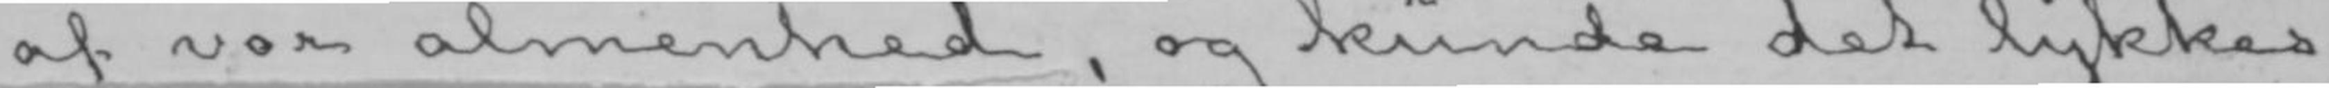af vor almenhed, og kunde det lykkes
